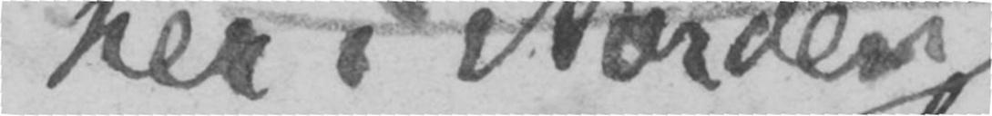her i Norden
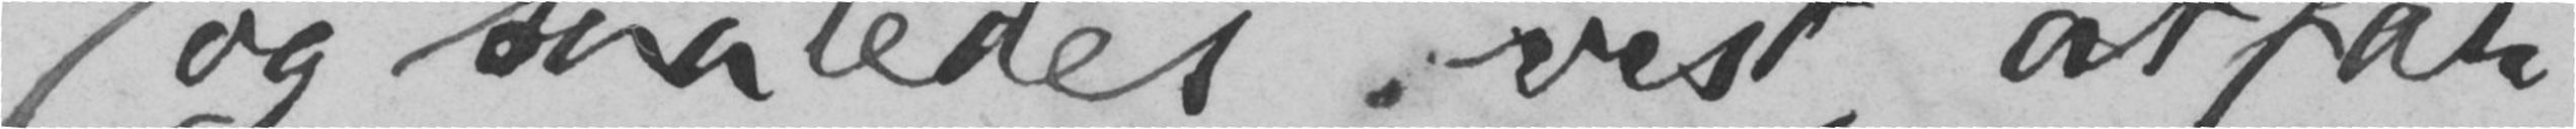og saaledes vist, at far-
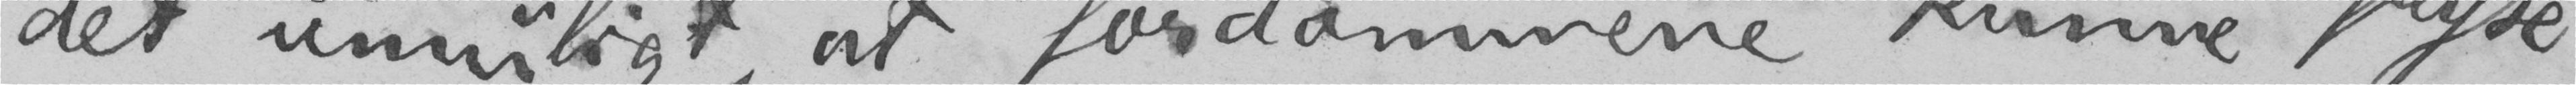det umuligt, at fordommene kunne fryse
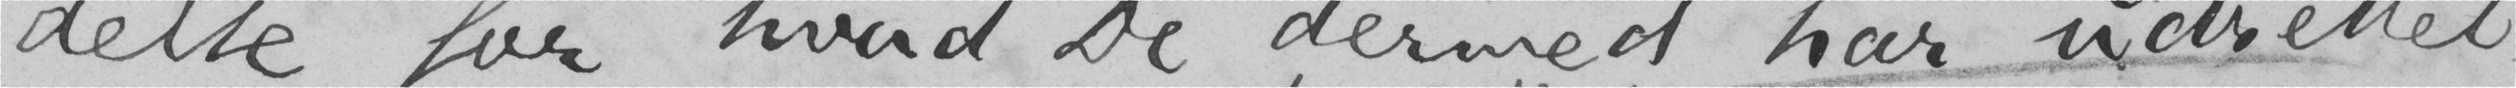delse for, hvad De dermed har udrettet.
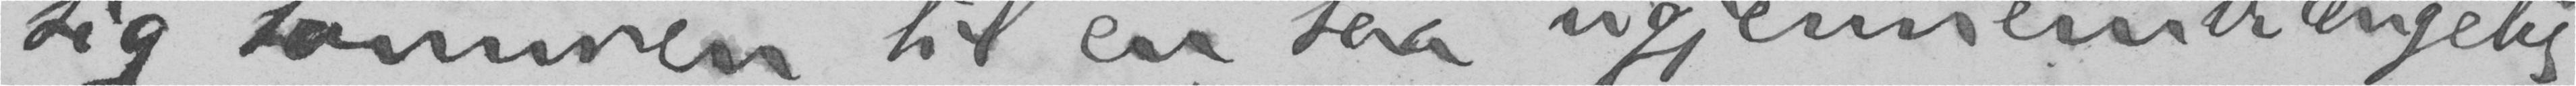sig sammen til en saa ugjennemtrængelig
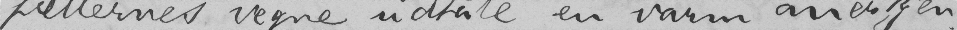fællernes vegne udtale en varm anerkjen-
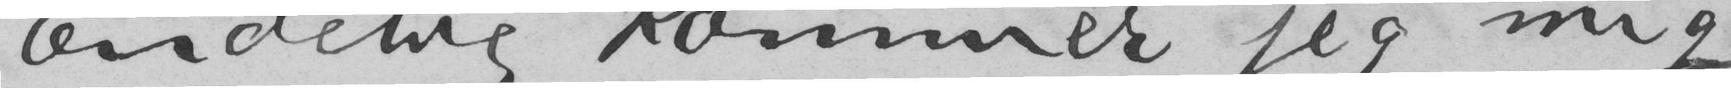Endelig kommer jeg mig
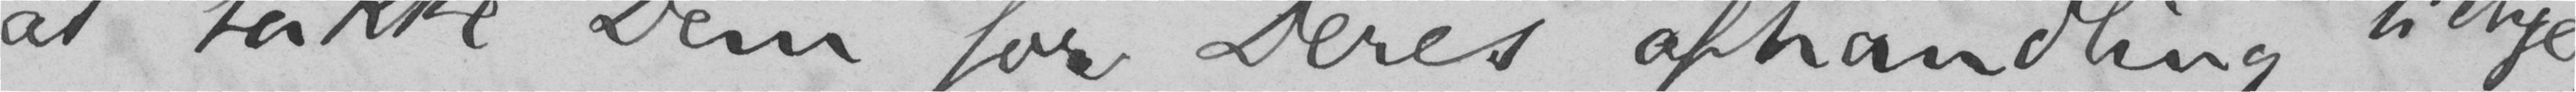at takke Dem for Deres afhandling tillige-
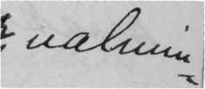ualmin-
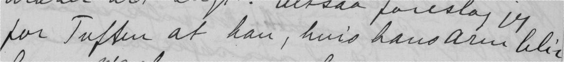for Tuften at han, hvis hans arm blir
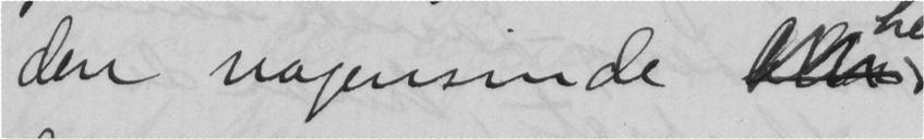den nogensinde
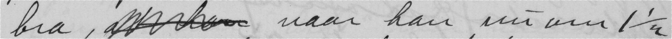bra,  naar han nu om 1 1/2
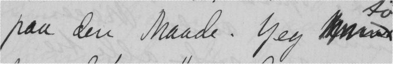paa den Maade. Jeg
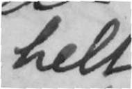helt
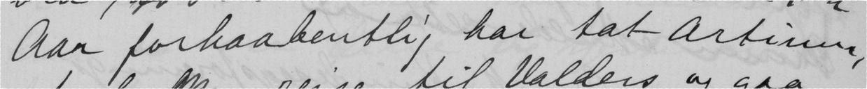Aar forhaabentlig har tat Artium,
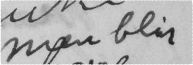men blir
